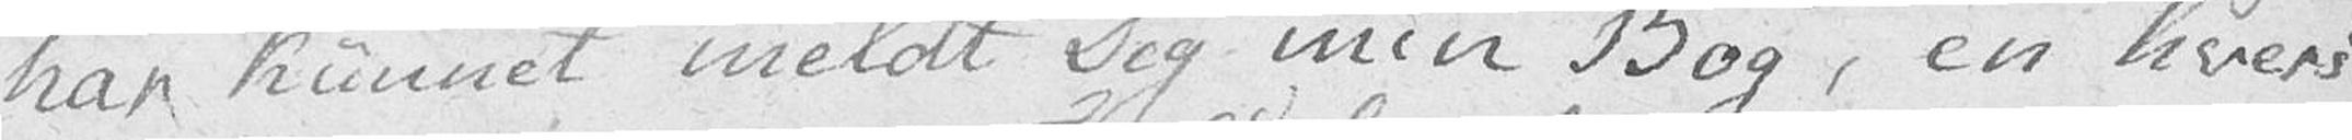har kunnet meldt Dig min Bog, en hvers
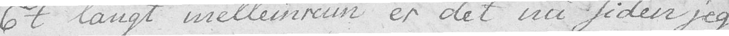Et langt mellemrum er det nu siden jeg
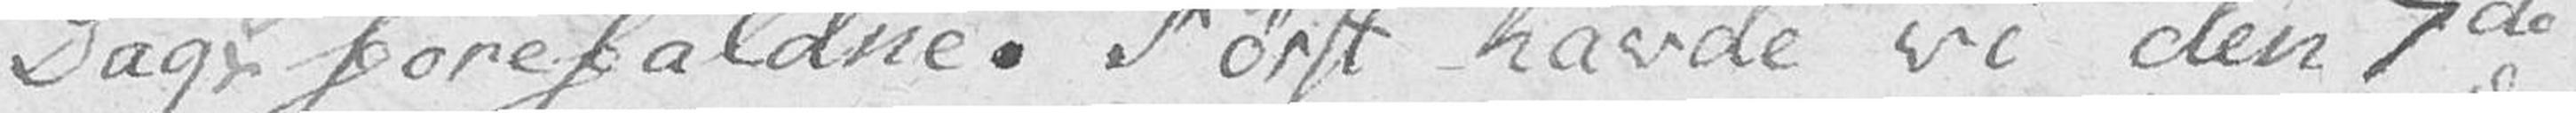Dags forefaldne. Først havde vi den 7de
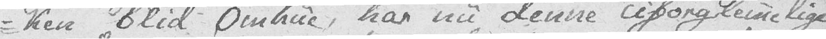-ken blid Omhue, har nu denne Uforglemmelige
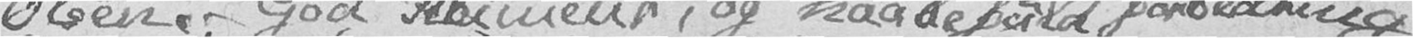Olsen. God Humeur, og haabefuld forbedring
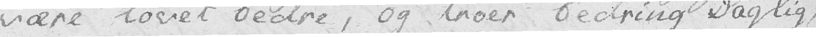være lovet bedre, og troer bedring Daglig,
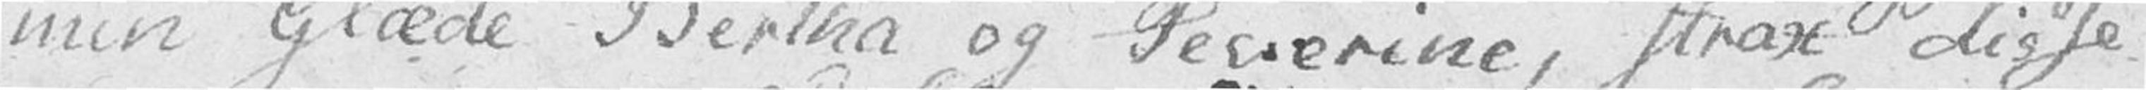min Glæde Bertha og Severine, strax disse
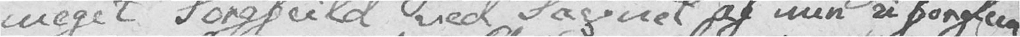meget Sorgfuld ved Savnet af min uforglem
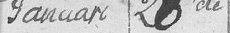Januari 26de
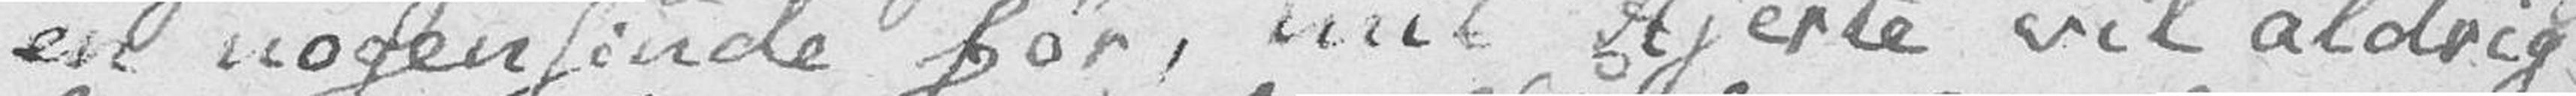en nogensinde før, mit Hjerte vil aldrig
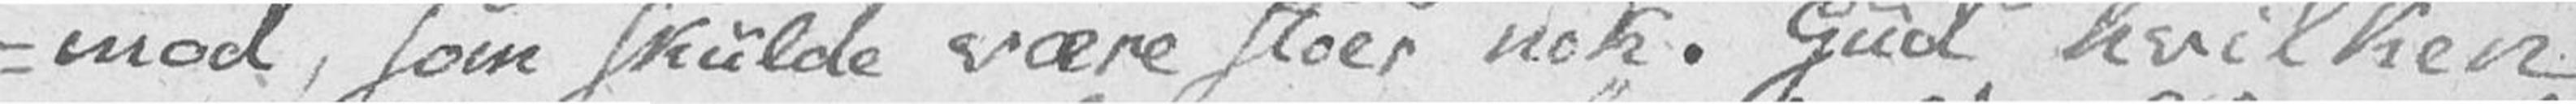-mod, som skulde være stoer nok. Gud hvilken
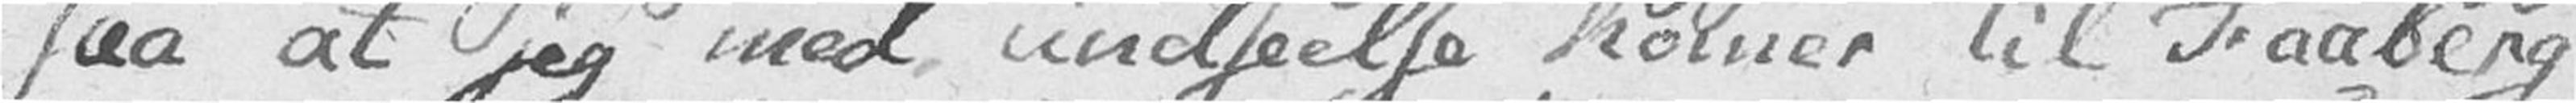saa at jeg med undseelse kommer til Faaberg
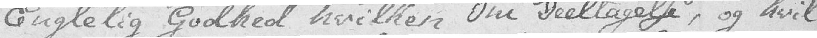Englelig Godhed hvilken Øm Deeltagelse, og hvil
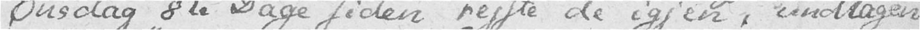Onsdag 8te Dage siden reiste de igjen, undtagen
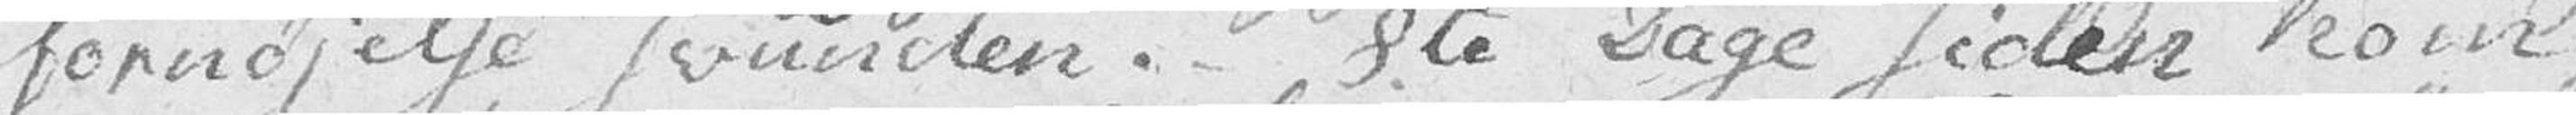fornøjelse svunden. 8te Dage siden kom
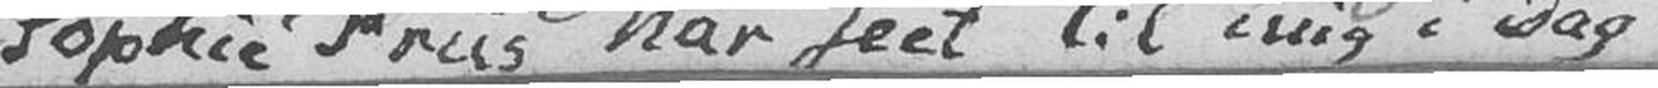Sophie Friis har seet til mig i Dag.
